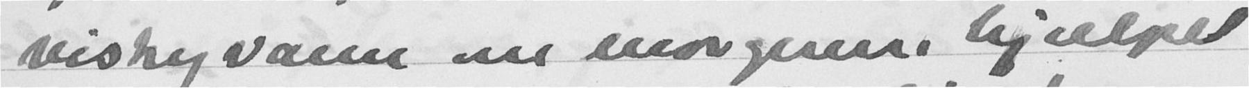vishyvann om morgenen hjelpet
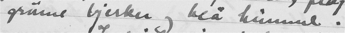grønne bjerker og blå himmel.
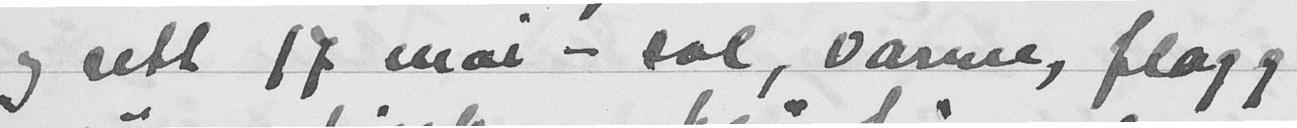og rett 17 mai - sol, varme, flagg
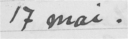17 mai.
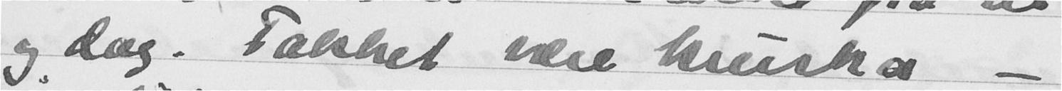og dag. Takket være kniske -
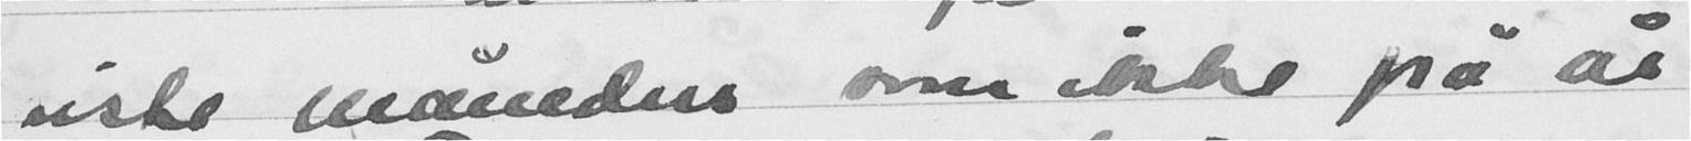siste måneden, som ikke på år
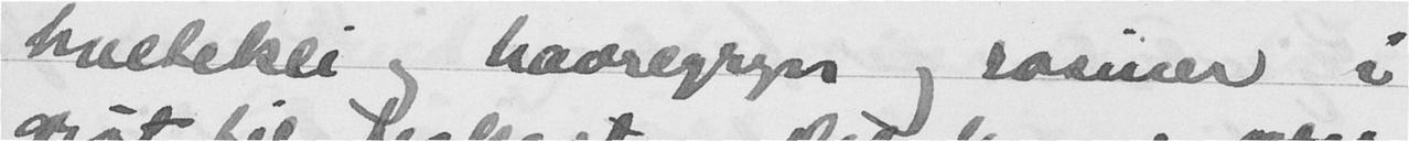buetekle og havregryn og rosiner i
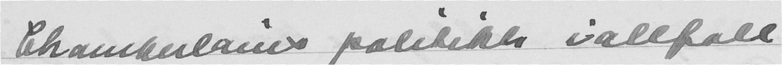Charlemaines politikk iallfall
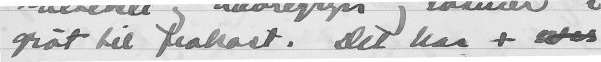grøt til frokost. Det har +
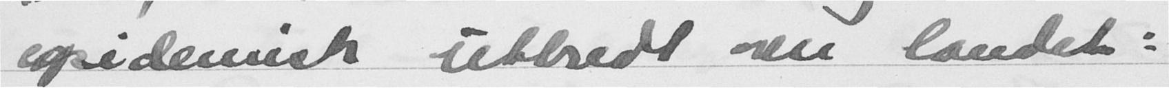epidemisk utbredt over landet:
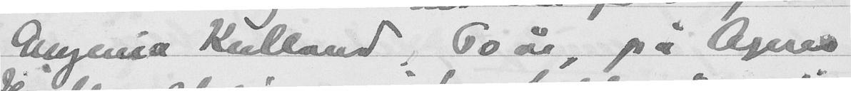Eugenia Kielland, 60 år, på Agnes
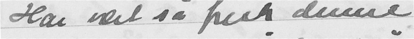Har vært så frisk denne
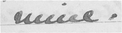mine.
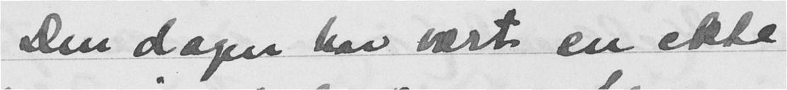Den dagen har vært en ekte
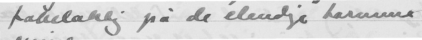fabelaktig på de elendige tarmene
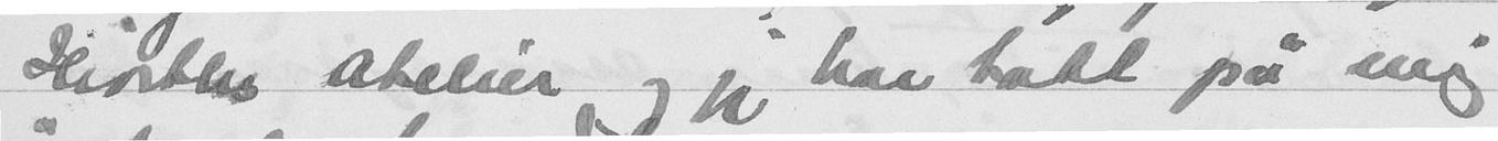Hiorths atelier og jeg har tatt på mig
